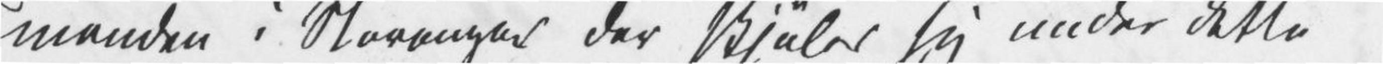manden i Stavanger der skjuler sig under dette
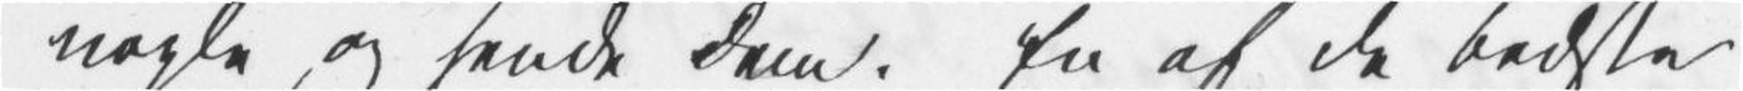nogle og sende Dem. En af de bedste
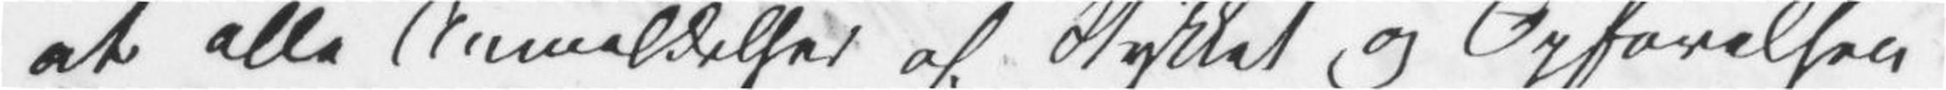at alle Anmeldelser af Stykket og Opførelser
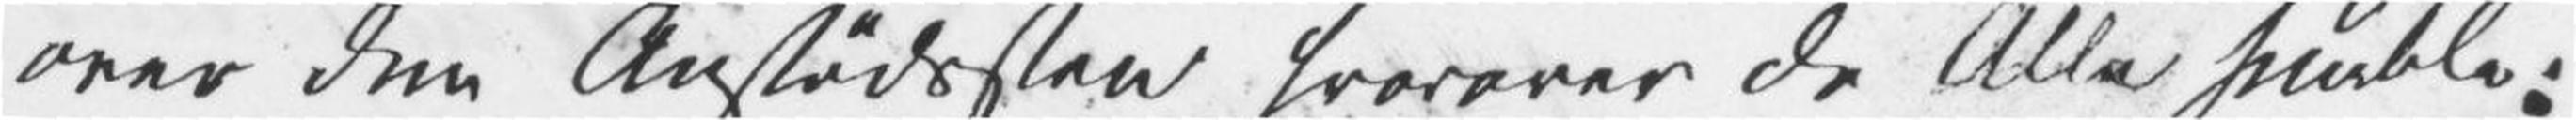over den Anstødssten hvorover de Alle snuble:
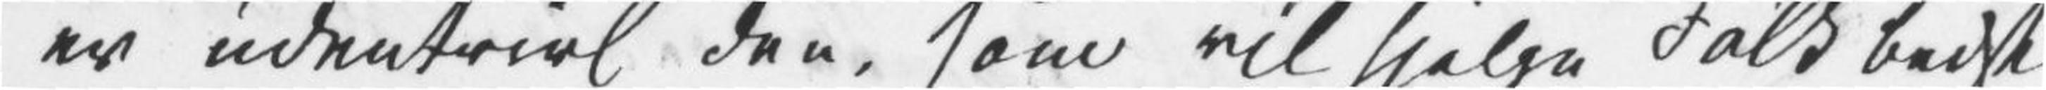er udentvivl den, som vil hjelpe Folk bedst
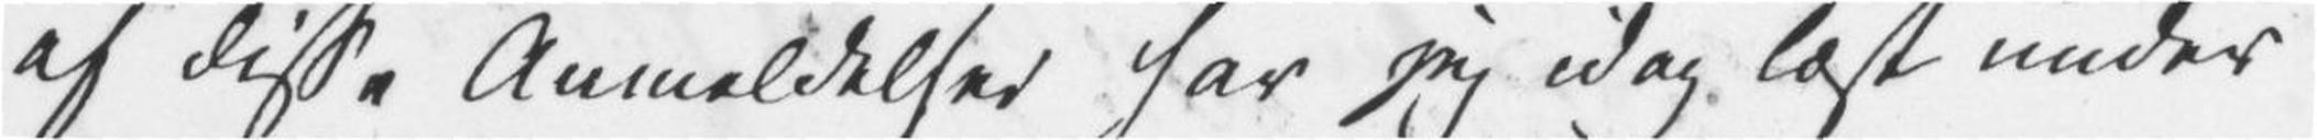af disse Anmeldelser har jeg idag læst under
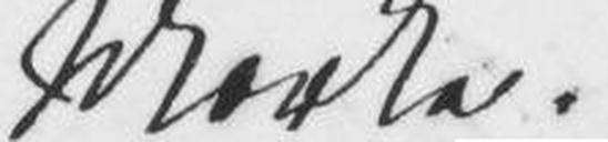Mærke.
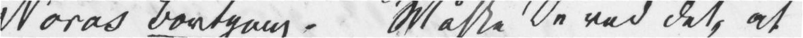Noras Bortgang. Måske De ved det, at
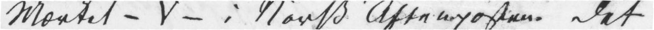Mærket – V – i Norsk «Aftenposten». Det
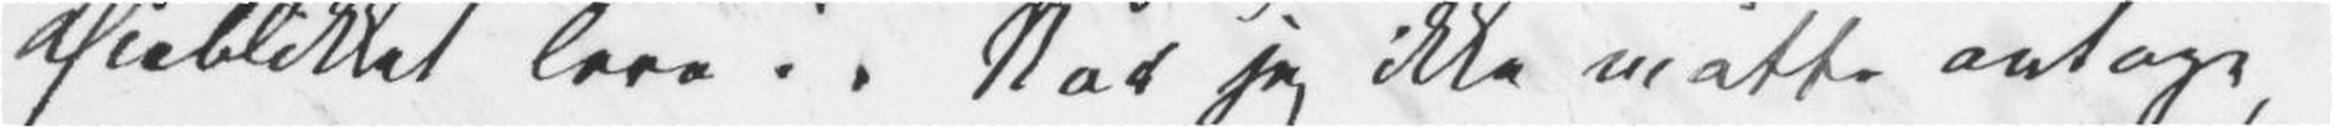Øieblikket leve i. Når jeg ikke måtte antage,
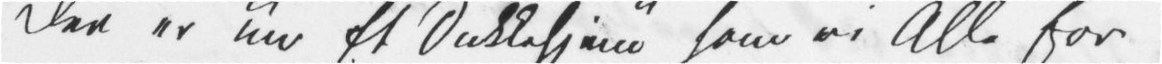Der er nu «Et Dukkehjem» som vi Alle for
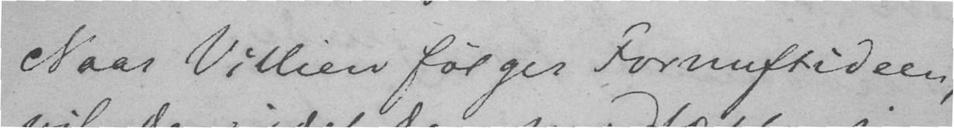Naar Villien følger Fornuftideen,
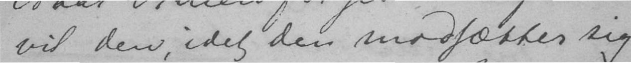vil den, idet den modsætter sig
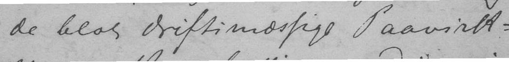de blot driftsmæssige Paavirk-
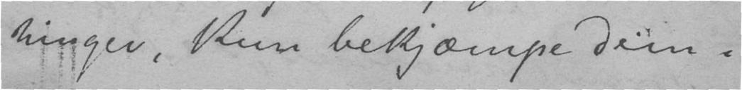ninger, kun bekjæmpe dem i
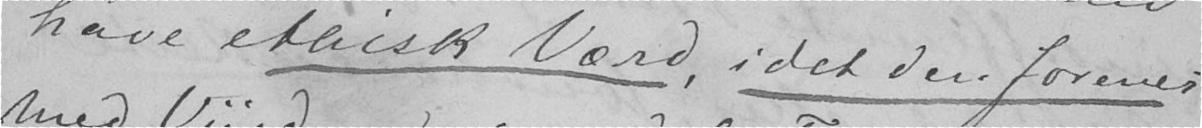have ethisk Værd, idet den forenes
